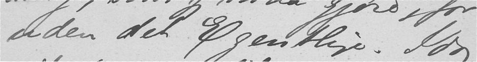uden det Egentlige. Idag
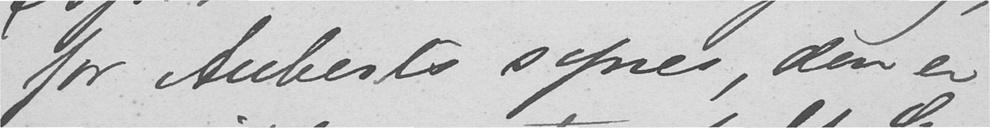for Auberts synes den er
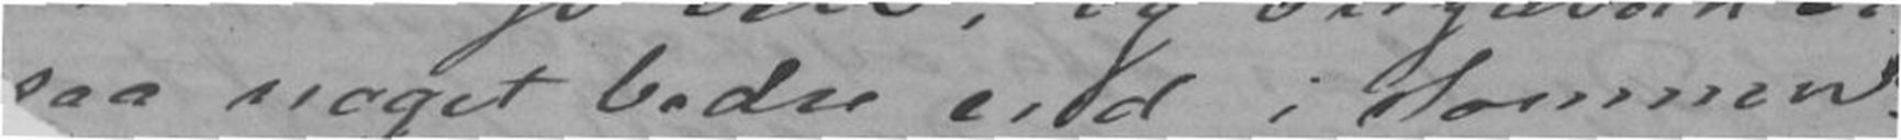saa noget bedre end i Sommer.
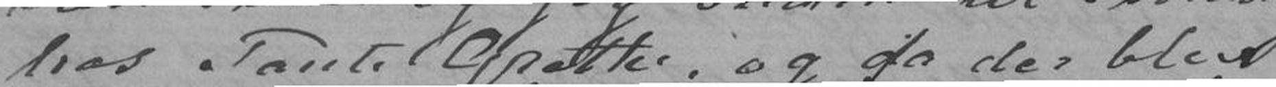hos Tante Grethe, og da der blev
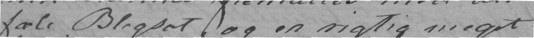fæle Blegsot og er rigtig meget
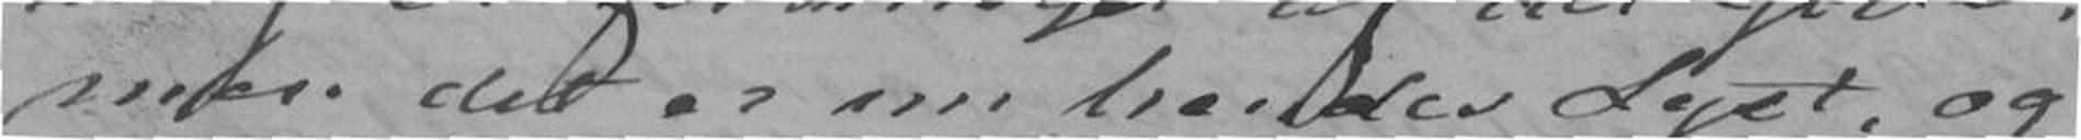men det er nu hendes Lyst, og
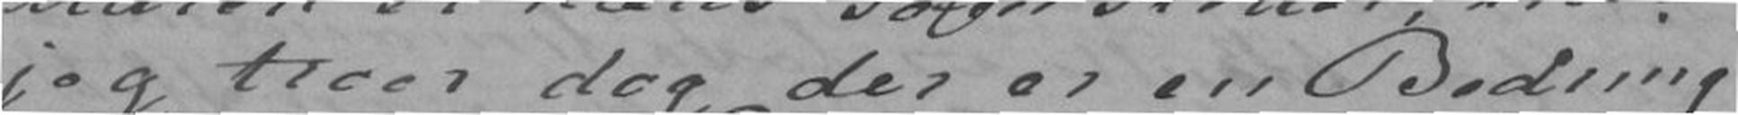jeg troer dog der er en Bedring
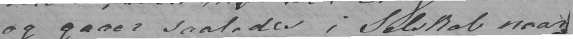og gaaer saaledes i Selskab naar
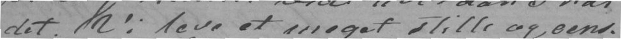det. Vi leve et meget stille og eens-
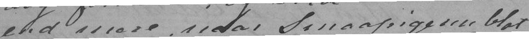end mere naar Smaapigerne blot
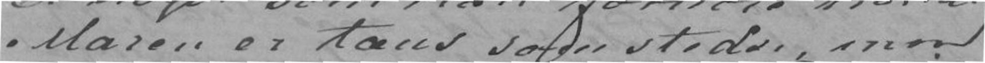Maren er taus som stedse, men
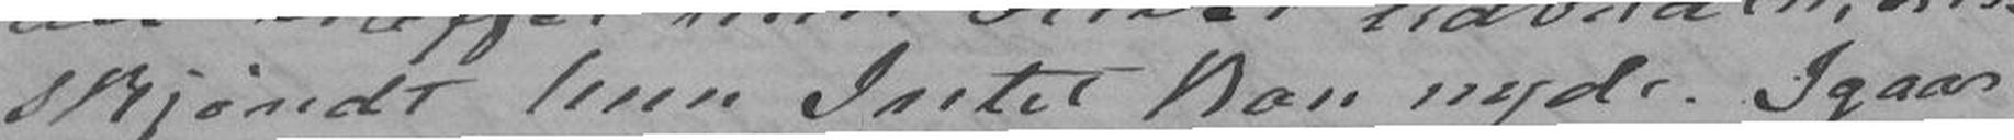skjøndt hun Intet kan nyde. Igaar
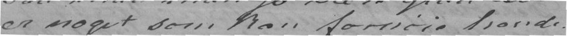er noget som kan fornøie hende.
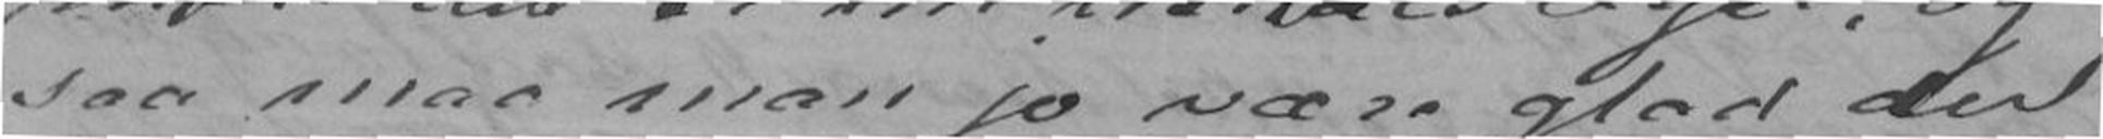saa maa man jo være glad der
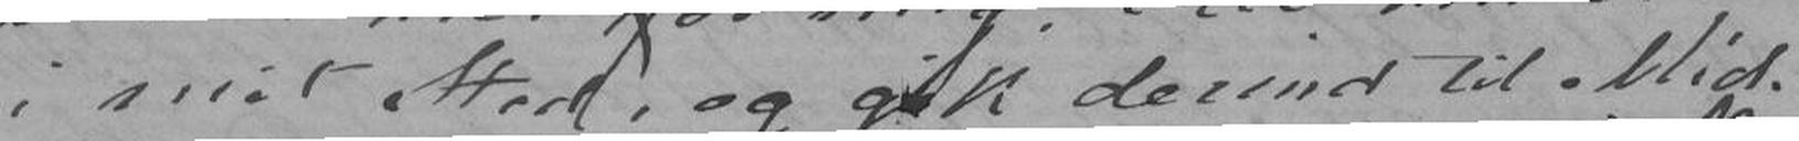i mit Sted, og gik derind til Mid-
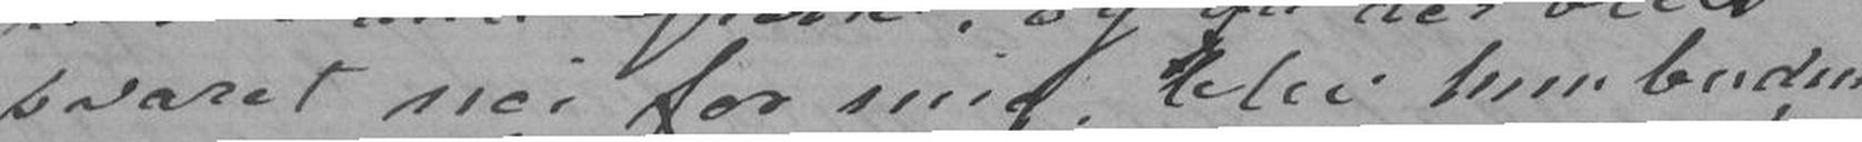svaret nei for mig, blev hun buden
